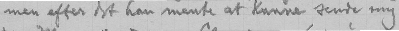men efter det han mente at kunne sende mig
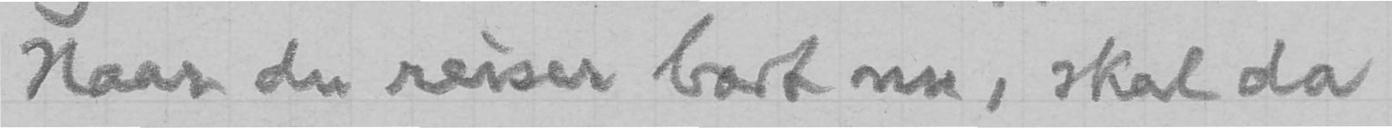Naar du reiser bort nu, skal da
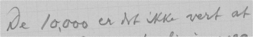De 10.000 er det ikke vert at
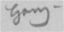Gang.
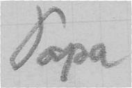Papa
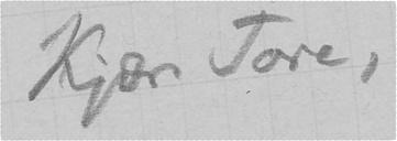Kjære Tore,
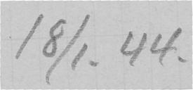18/1. 44.
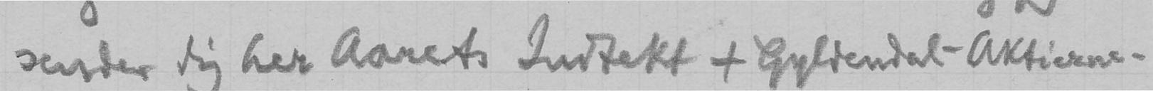sender dig her Aarets Indtekt + Gyldendal-Aktierne.
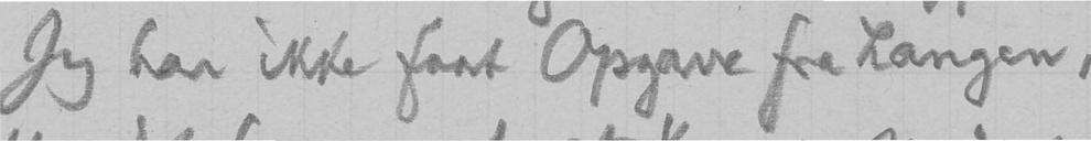Jeg har ikke faat Opgave fra Langen,
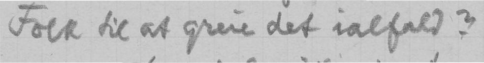Folk til at greie det ialfald?
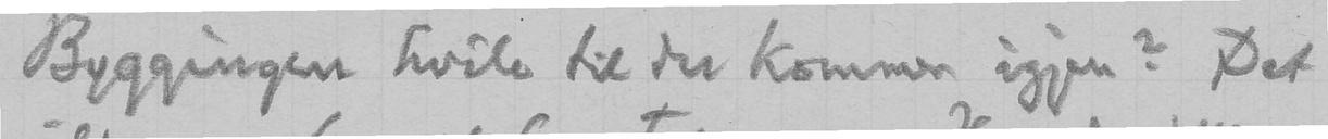Byggingen hvile til du kommer igjen? Det
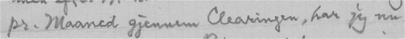pr. Maaned gjennem Clearingen, har jeg nu
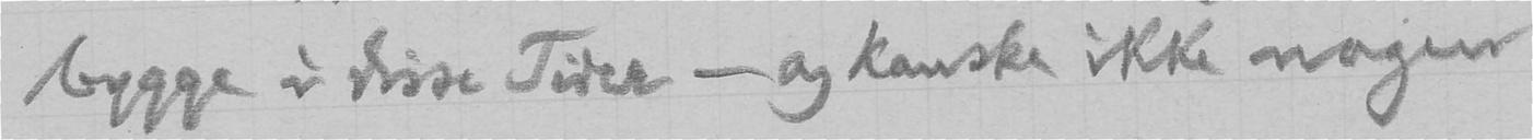bygge i disse Tider - og kanske ikke nogen
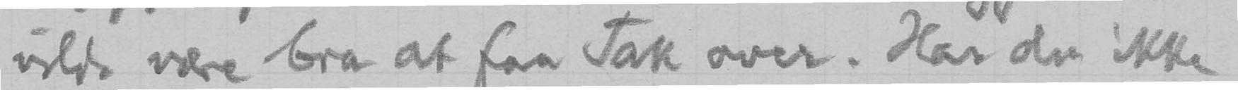vilde være bra at faa Tak over. Har du ikke
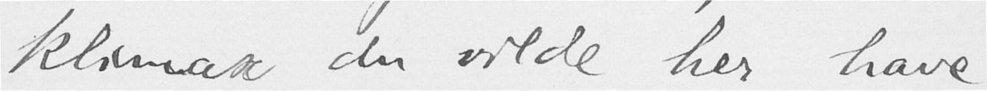klimax, du vilde her have
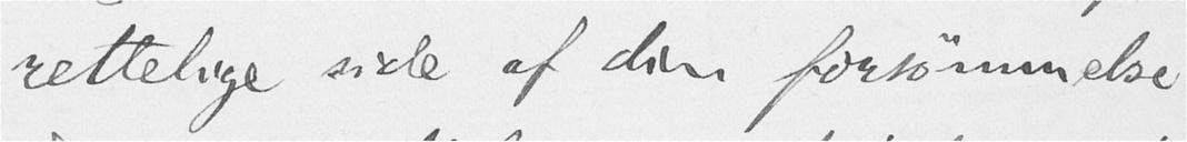rettelige side af din forsømmelse.
